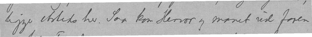ligger etsteds her. Saa kom Hervor og manet ud faren
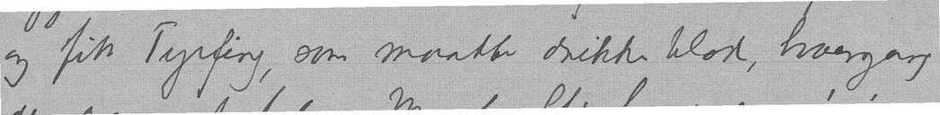og fik Tyrfing, som maatte drikke blod, hvergang
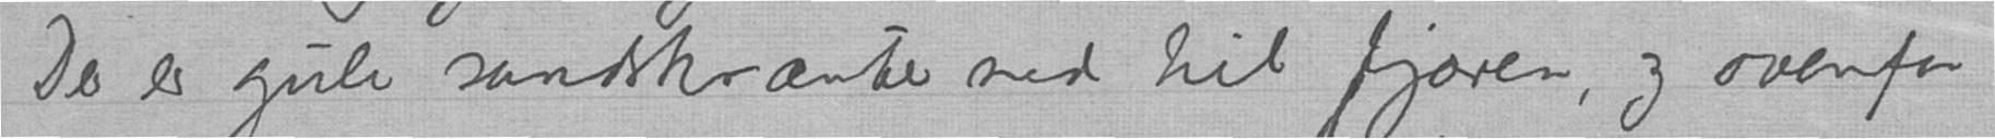Der er gule sandskrænter ned til fjoren, og ovenfor
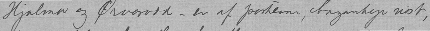Hjalmar og Ørverodd - en af parterne, Angantyr vist,
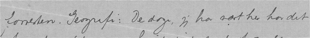forresten. Geografi: De dage, jeg har vært her har det
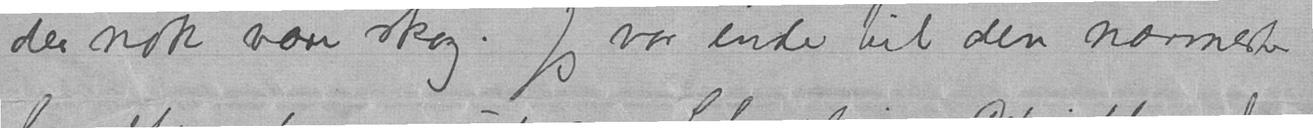der nok være skog. Jeg var inde til den nærmeste
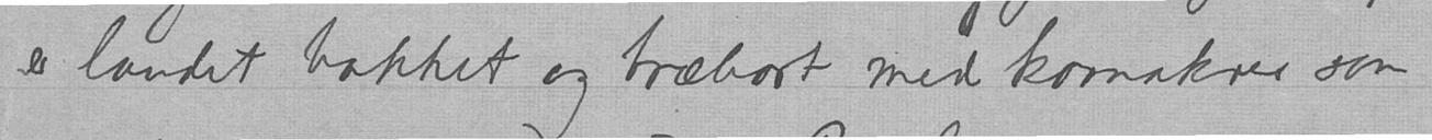er landet bakket og træbart med kornakrer som
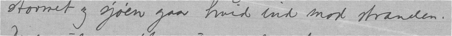stormet og sjøen gaar hvid ind mod stranden.
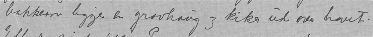bakkerne ligger en gravhaug og kiker ud over havet.
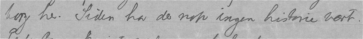borg her. Siden har der nok ingen historie vært.
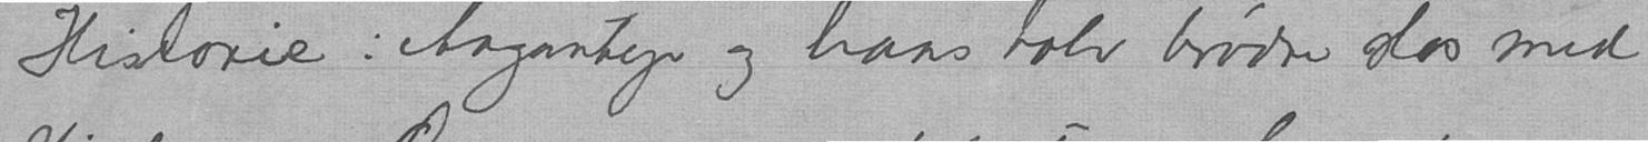Historie: Angantyr og hans tolv brødre slos med
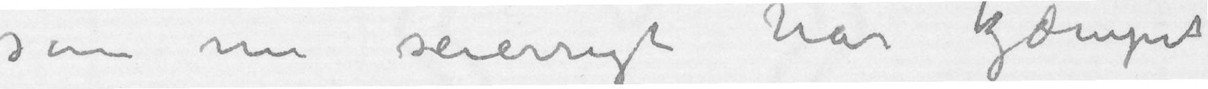som nu seierrigt har kjæmpet
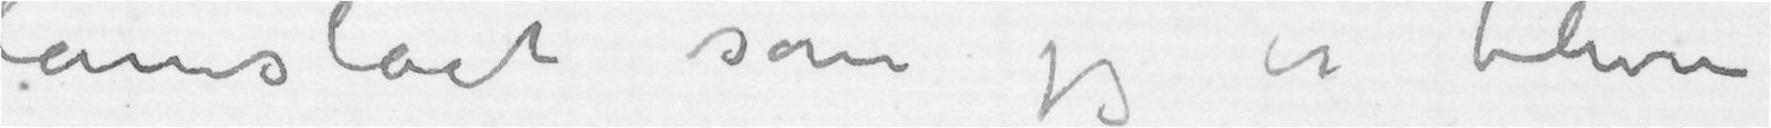lamslået som jeg er bleven
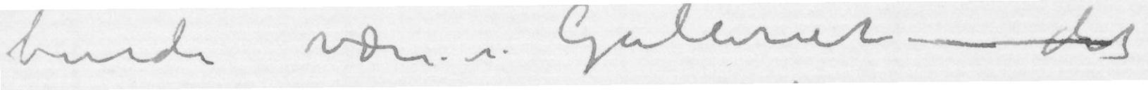burde være i Galleriet – det
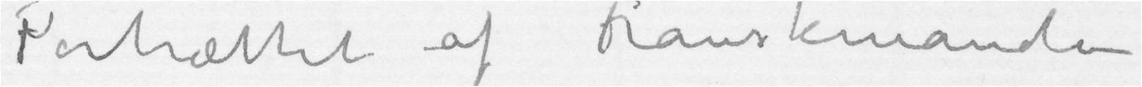Portrættet av Franskmanden
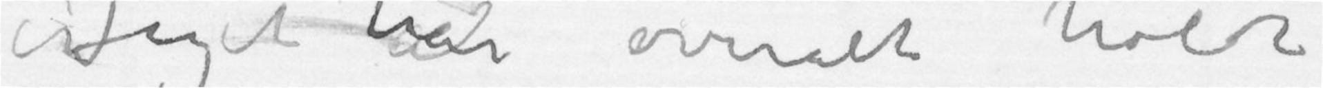er et aJeg har overalt holdt
